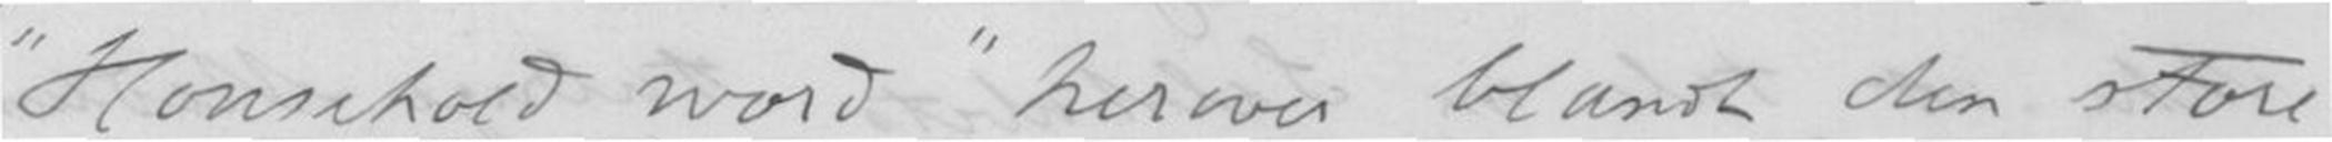"Household word" herover blandt den store
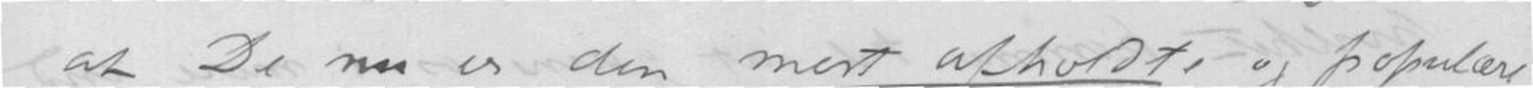at De nu er den mest afholdte og populær
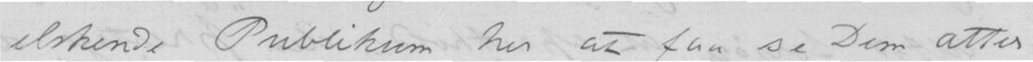elskende Publikum her at faa se Dem atter
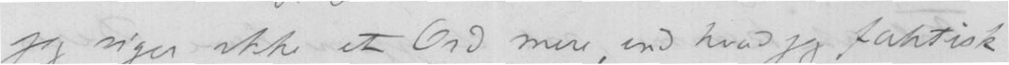jeg siger ikke et Ord mere, end hvad jeg faktisk
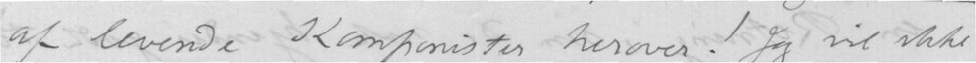af levende Komponister herover! Jeg vil ikke
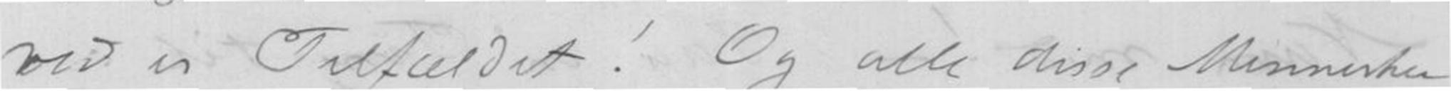ved er Tilfældet! Og alle dissse Mennesker
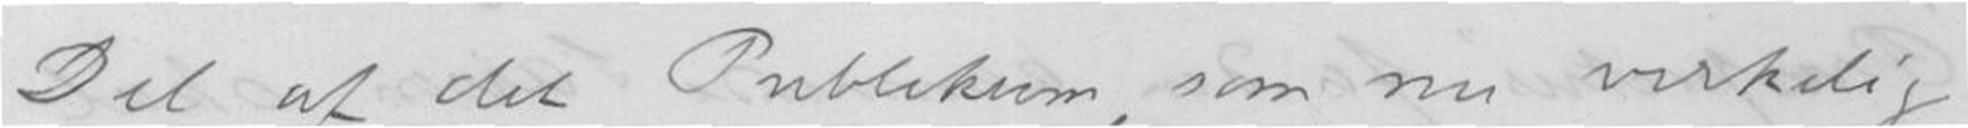Del af det Publikum, som nu virkelig
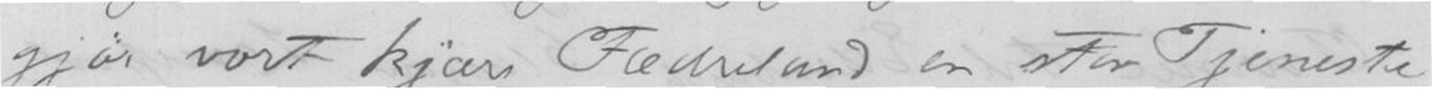gjør vort kjære Fædreland en stor Tjeneste,
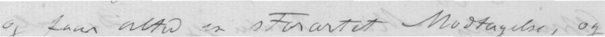og faar altid en stoartet Modtagelse, og
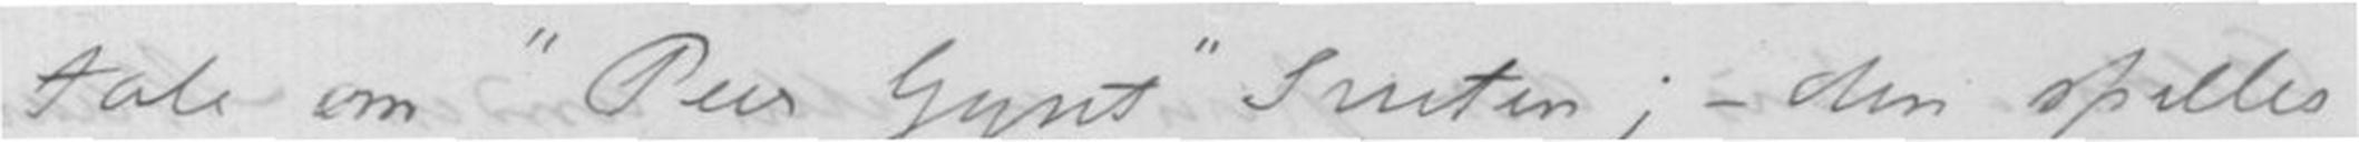tale om "Peer Gynt" Suiten; - den spilles
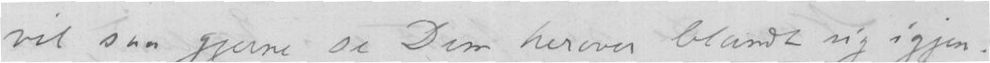vil saa gjerne se Dem herover blandt sig igjen!
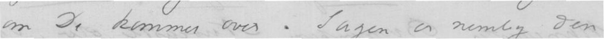om De kommer over. Sagen er nemlig den
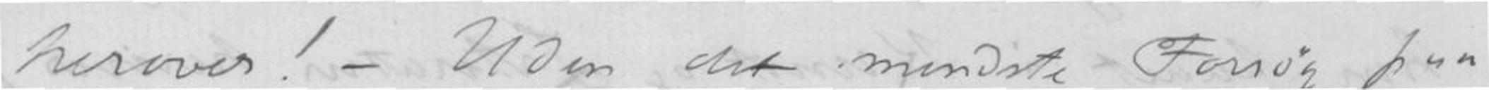herover! - Uden det mindste Forsøg paa
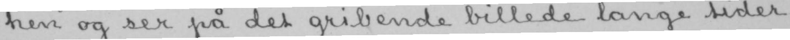hen og ser på det gribende billede lange tider
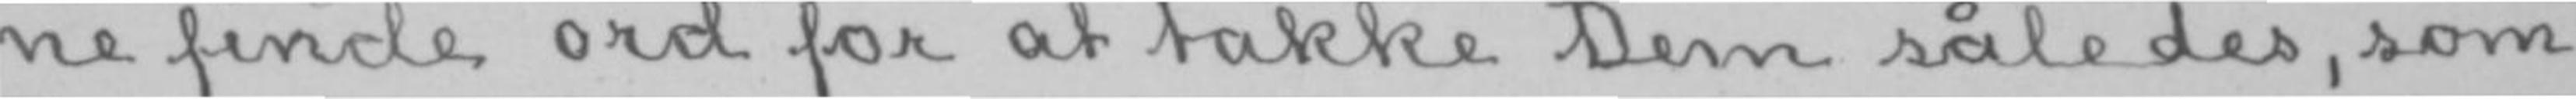ne finde ord for at takke Dem således, som
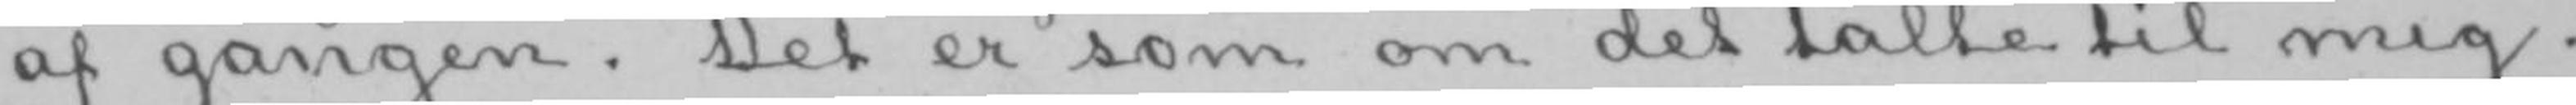af gangen. Det er som om det talte til mig.
